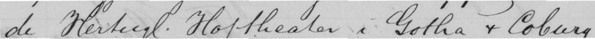de Hertugl Hoftheater i Gotha & Coburg
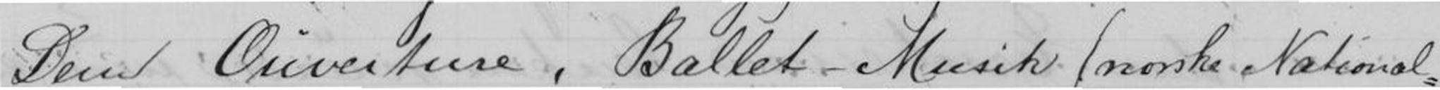Dem Ouverture, Ballet Musik (norske National-
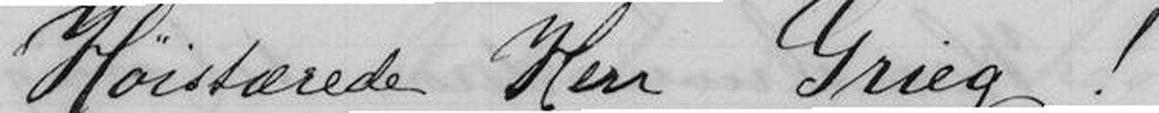Høistærede Herr Grieg!
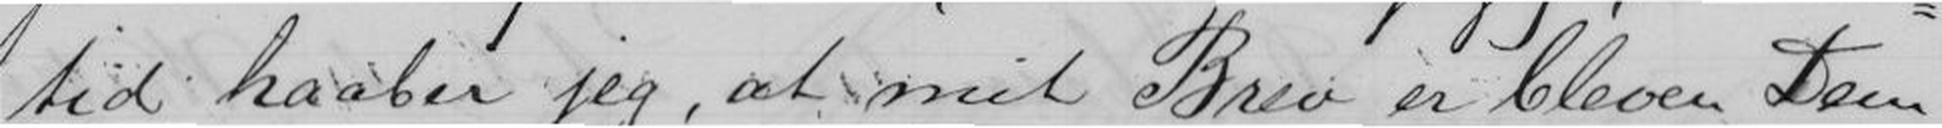tid haaber jeg, at mit Brev er bleven Dem
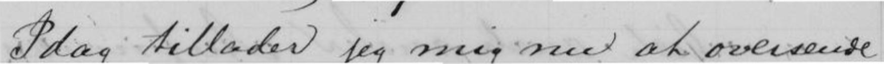Idag tillader jeg mig nu at oversende
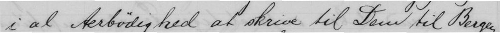i al Aerbødighed at skrive til Dem til Bergen
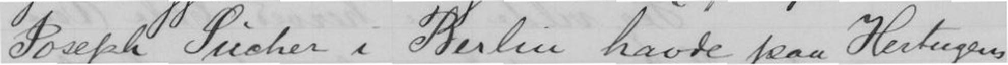Joseph Sucher i Berlin havde paa Hertugens
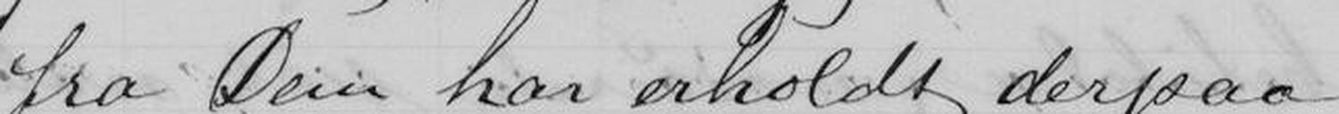fra Dem har erholdt derpaa.
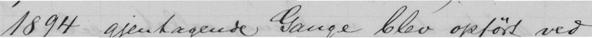1894 gjentagende Gange blev opført ved
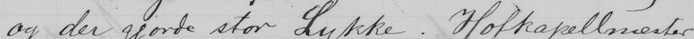og der gjorde stor Lykke. Hofkapellmester
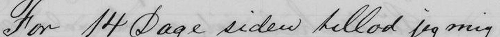For 14 Dage siden tillod jeg mig
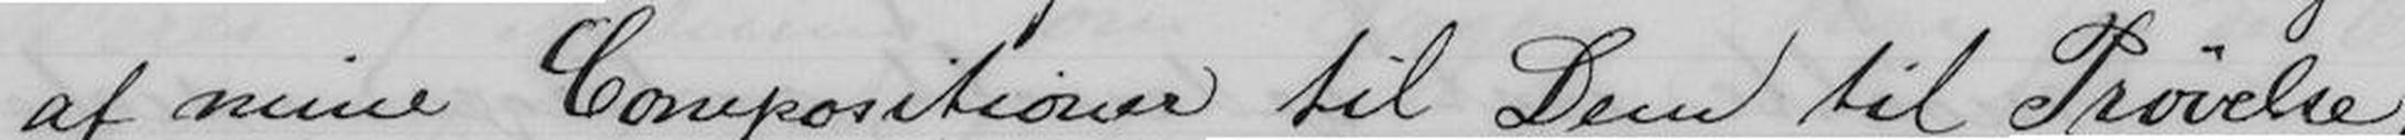af mine Compositioner til Dem til Prøvelse,
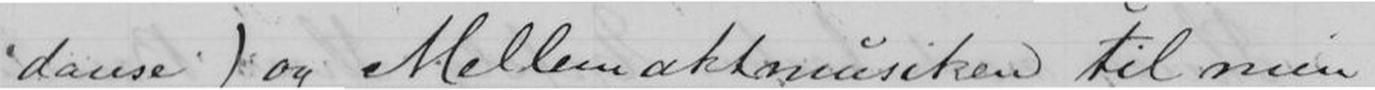danse) og Mellemaktmusiken til min
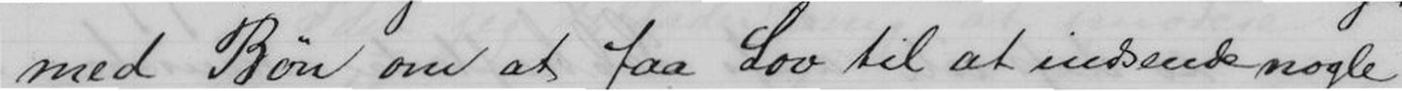med Bøn om at faa Lov til at indsende nogle
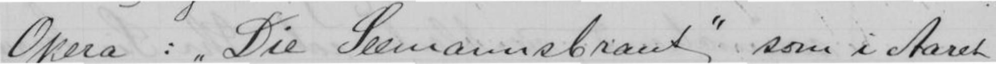Opera: Die Seemannsbraut som i Aaret
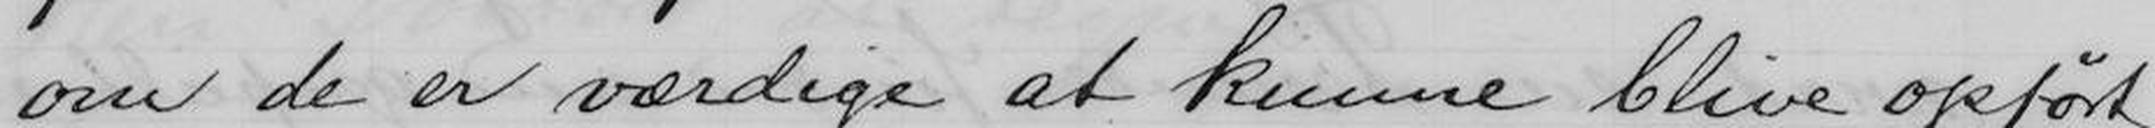om de er værdige at kunne blive opført
